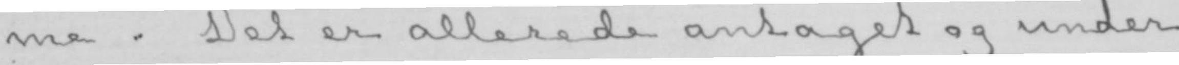me. Det er allerede antaget og under
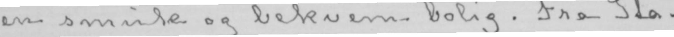en smuk og bekvem bolig. Fra Ita-
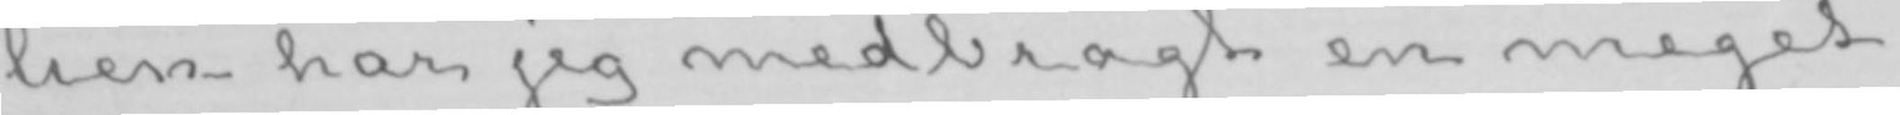lien har jeg medbragt en meget
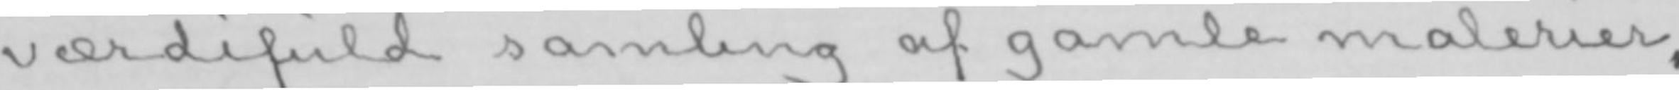værdifuld samling af gamle malerier,
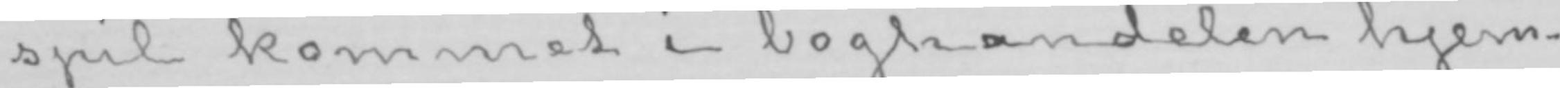spil kommet i boghandelen hjem-
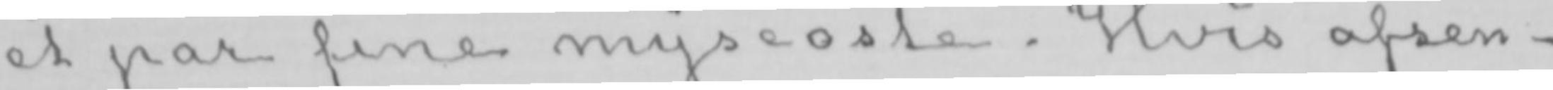et par fine myseoste. Hvis afsen-
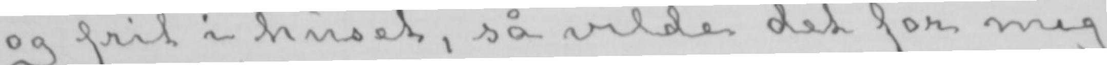og frit i huset, så vilde det for mig
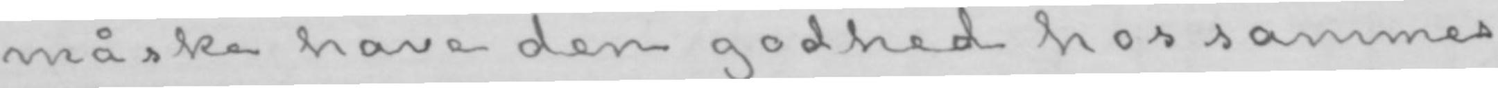måske have den godhed hos sammes
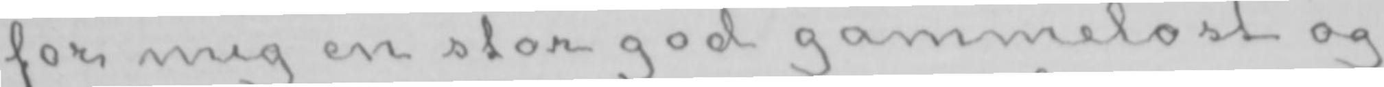for mig en stor god gammelost og
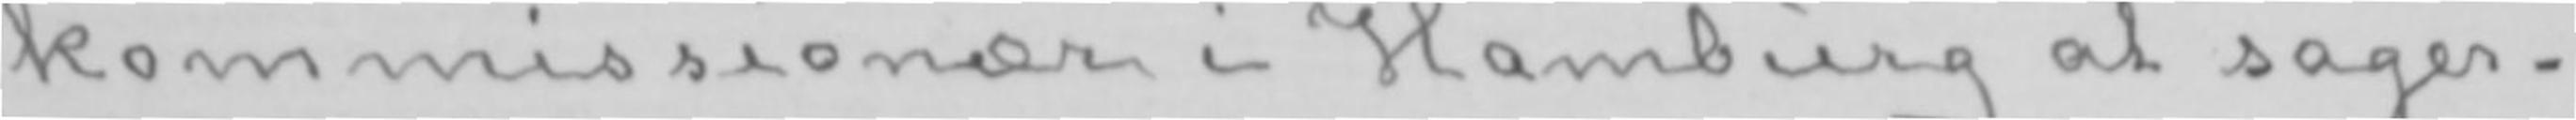kommissionær i Hamburg at sager-
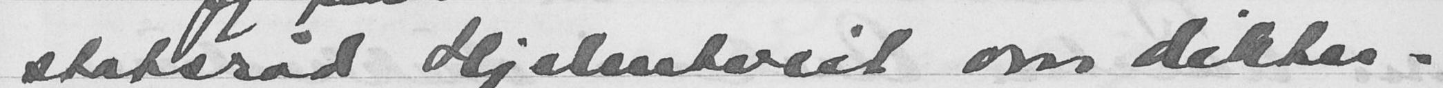statsråd Hjelmtveit om dikter-
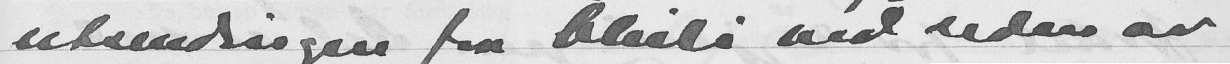utsendingen fra Bhili ved siden av
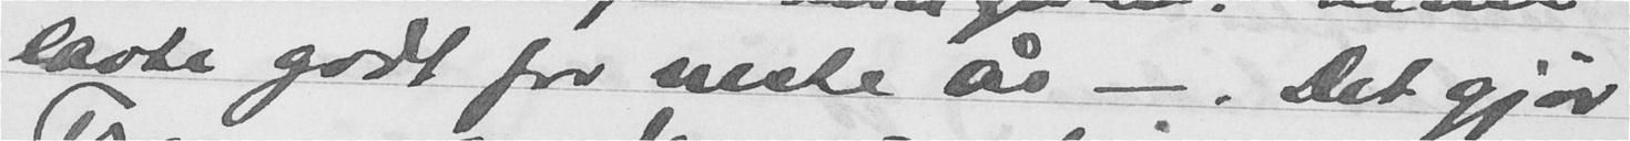lovte godt for neste år -. Det gjør
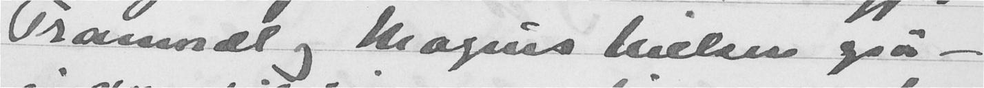Tranmæl og Magnus Nilssen også -
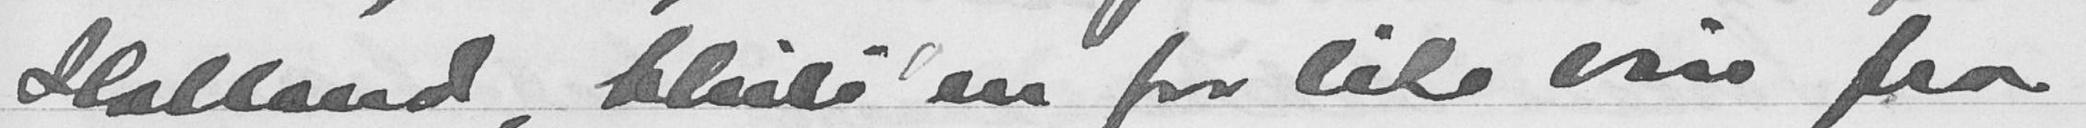Holland, Bhili'en for lite vin fra
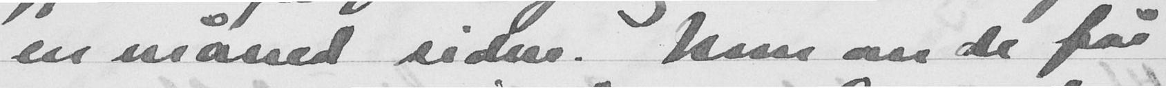en måned siden. Men om de får
